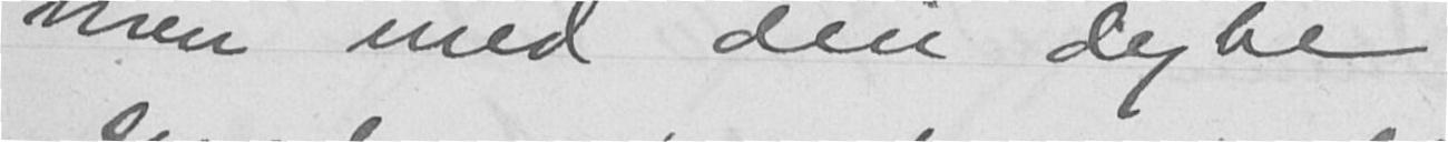men med den dybe
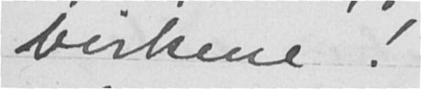birkene!
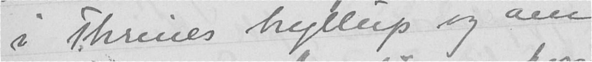i Thrines bryllup og om
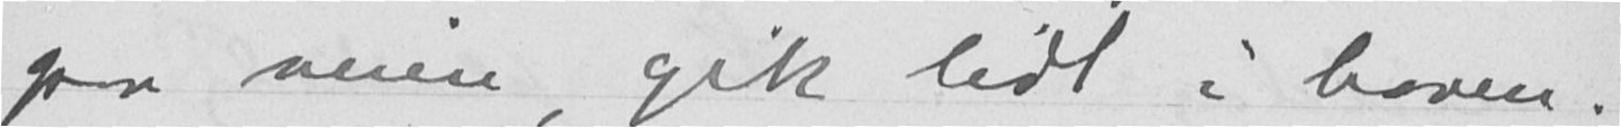paa veien, gik lidt i haven.
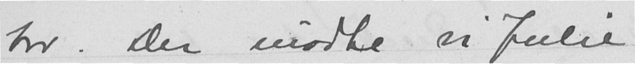bor. Der mødte vi Julie
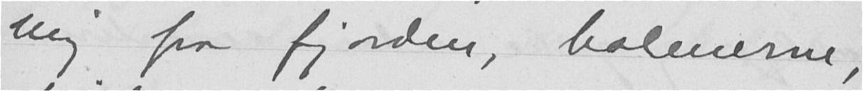mig fra fjorden, holmerne,
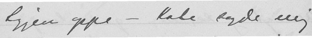Siggen oppe - Kate sagde mig
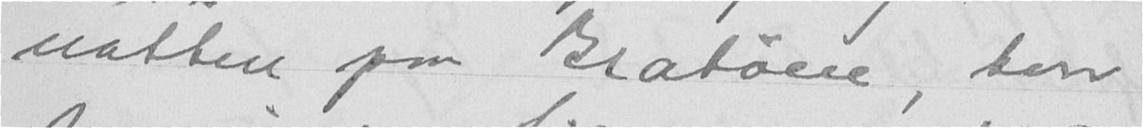natten paa Bratøen, hvor
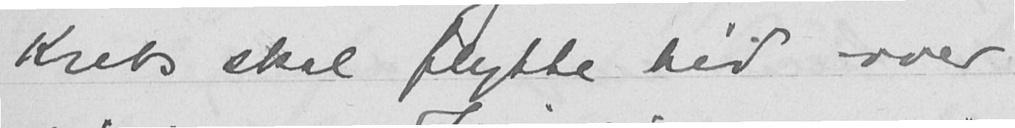Krebs skal flytte hid over
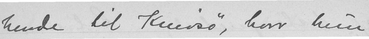hende til Knivsø, hvor hun
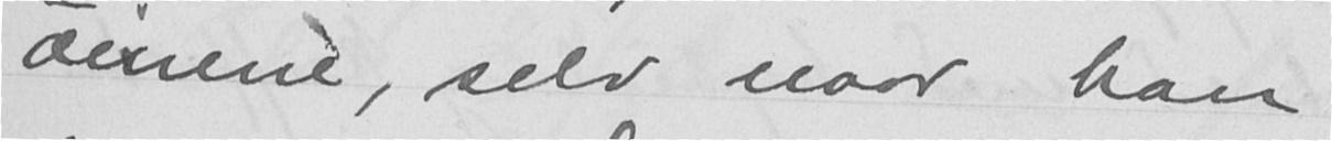øinene, selv naar han
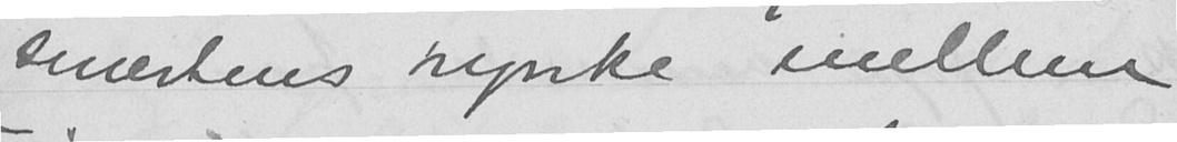smertens rynke mellem
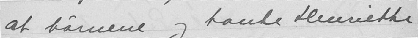at børnene og tante Henriette
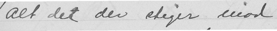Alt det der stiger mod
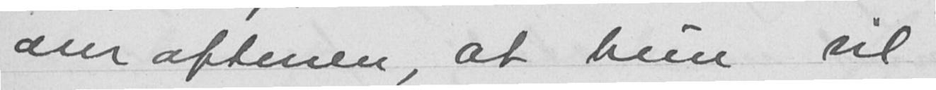om aftenen, at hun vil
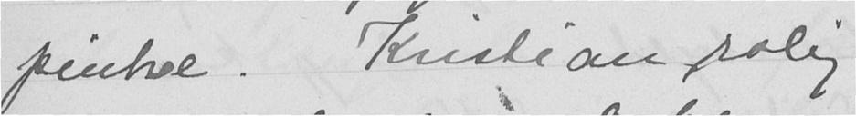pintse. Kristian rolig
